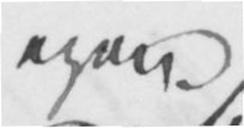egen
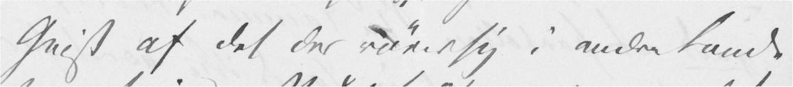Gnist af det der rører sig i andre Lande
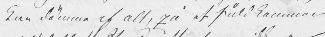kan dømme af alt, på et fuldkommen
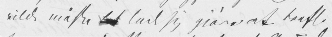vilde måske let lade sig gjøre at treffe
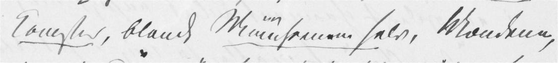komster, blandt Müncherne selv, Mændene,
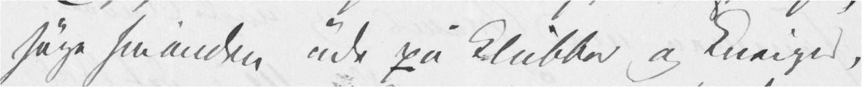søge hinanden ude på Klubber og Kneiper,
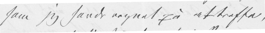som jeg havde regnet på at treffe,
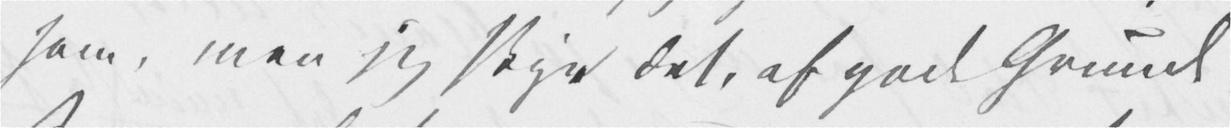ham, men jeg skyr det, af gode Grunde
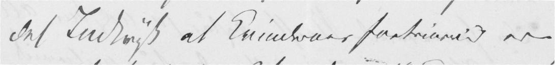det Indtryk at Kvindernes fortrinvis ere
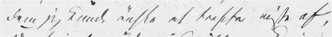dem jeg kunde ønske at treffe visse af,
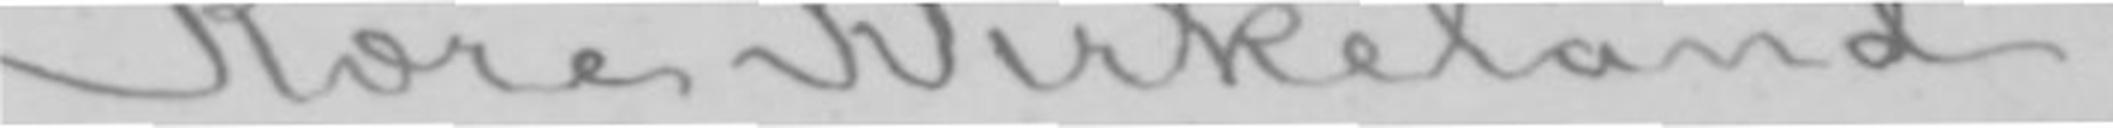Kære Birkeland!
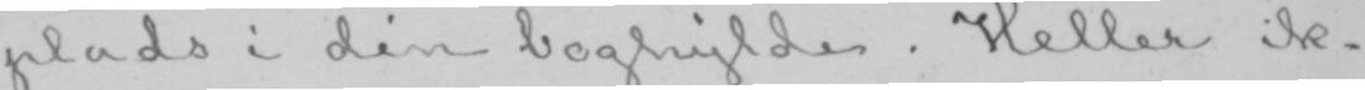plads i din boghylde. Heller ik-
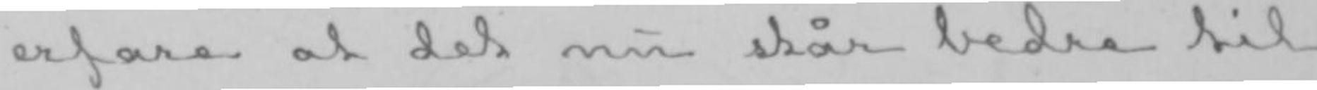erfare at det nu står bedre til
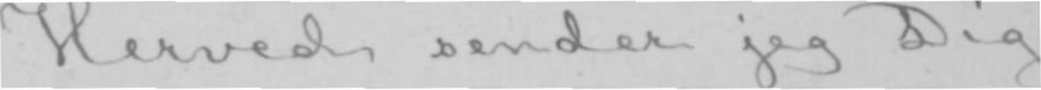Herved sender jeg Dig
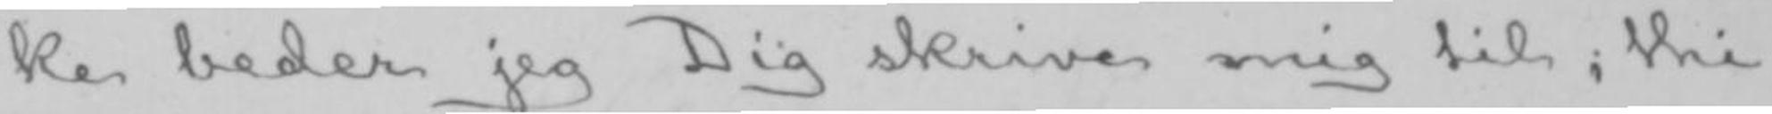ke beder jeg Dig skrive mig til; thi
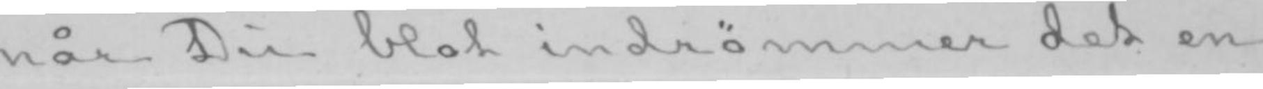når Du blot indrømmer det en
